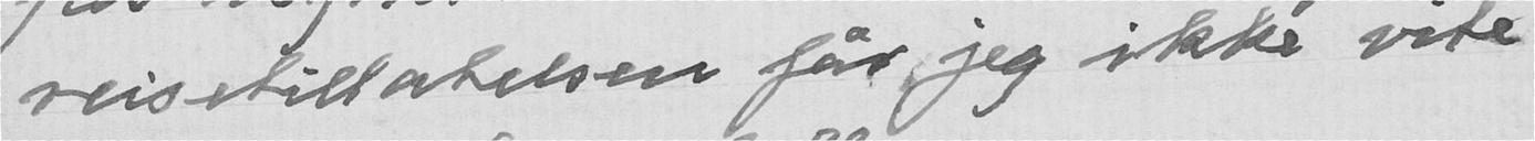reisetillatelsen får jeg ikke vite
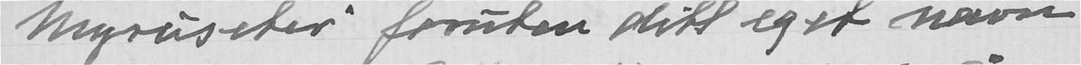Mysuseter foruten ditt eget navn
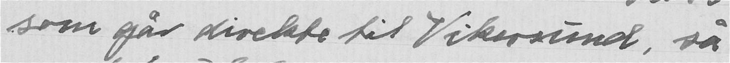som går direkte til Vikersund, så
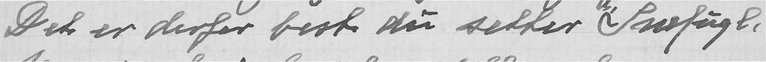Det er derfor best du setter Snefugl,
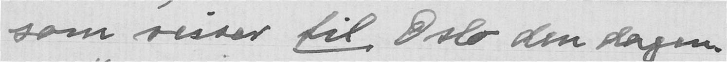som reiser til Oslo den dagen.
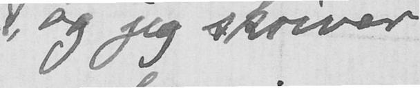og jeg skriver
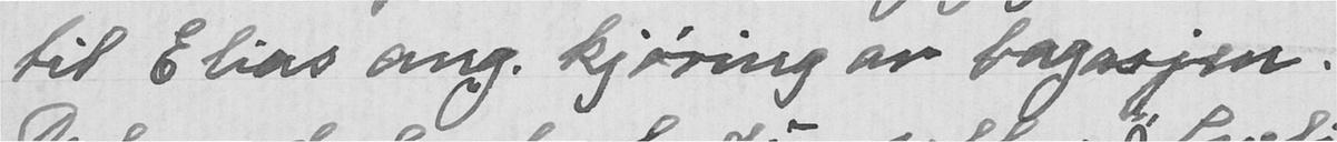til Elias ang. kjøring av bagasjen.
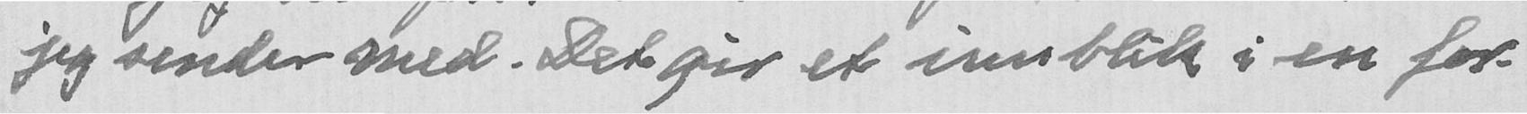jeg sender med. Det gir et innblik i en for-
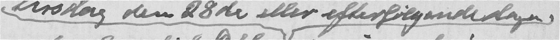tirsdag den 28de eller efterfølgende dage,
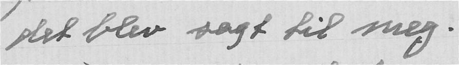det blev sagt til meg.
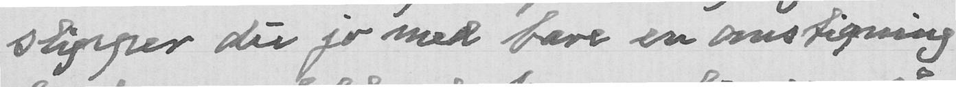slipper du jo med bare en omstigning
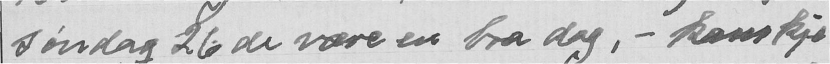søndag 26de være en bra dag, - kanskje
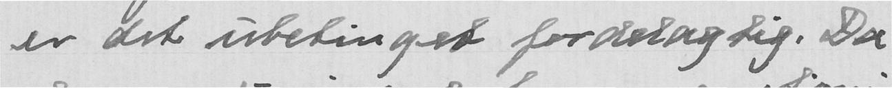er det ubetinget fordelagtig. Da
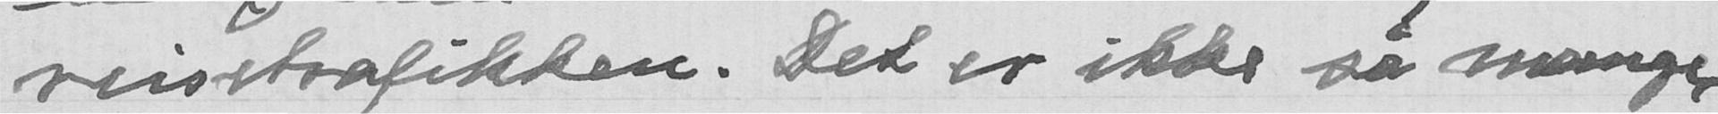reisetrafikken. Det er ikke så mange
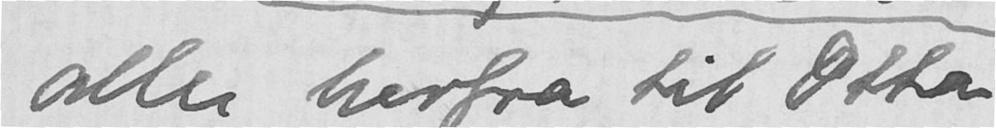alle herfra til Otta,
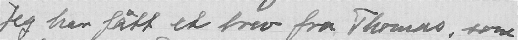Jeg har fått et brev fra Thomas, som
